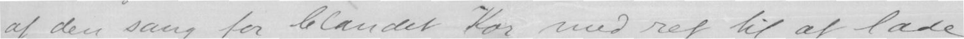af den sang for blandet Kor med ret til at lade
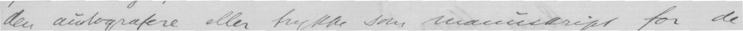den autografere eller trykke som manuskript for de
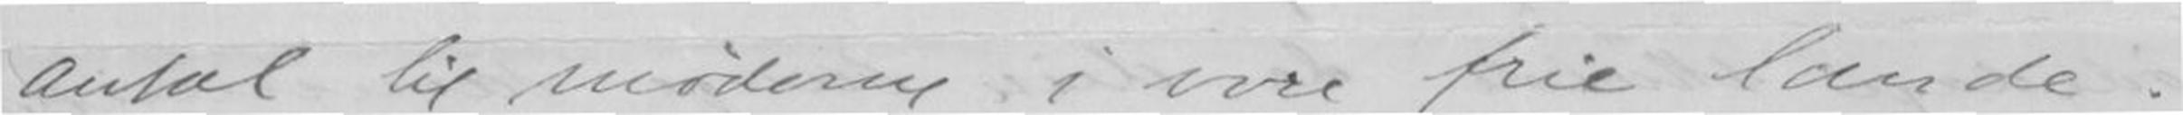antal til møderne i vore frie lande.
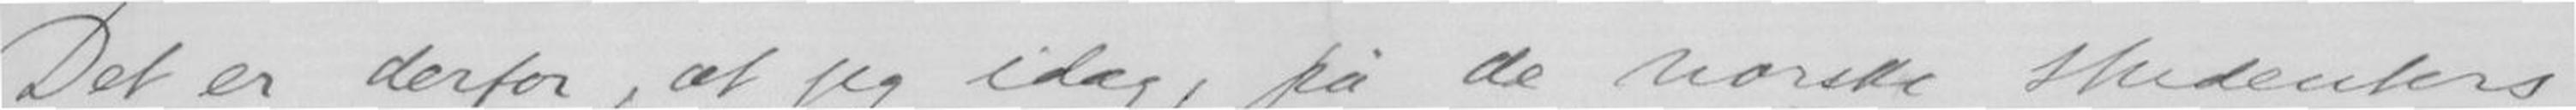Det er derfor, at jeg idag, på de norske studenters
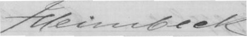J. Heimbeck
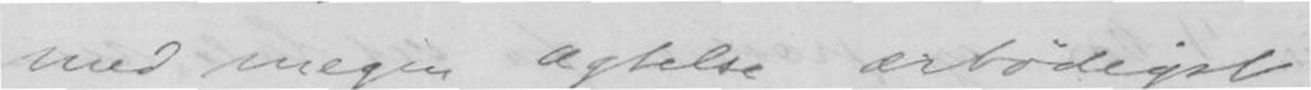Med megen agtelse ærbødigst
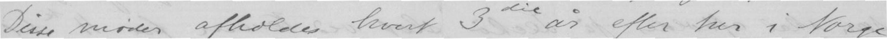Disse møder afholdes hvert 3die år efter tur i Norge
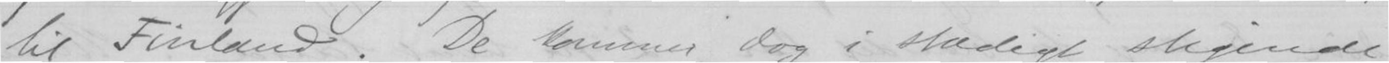til Finland. De kommer dog i stadigt stigende
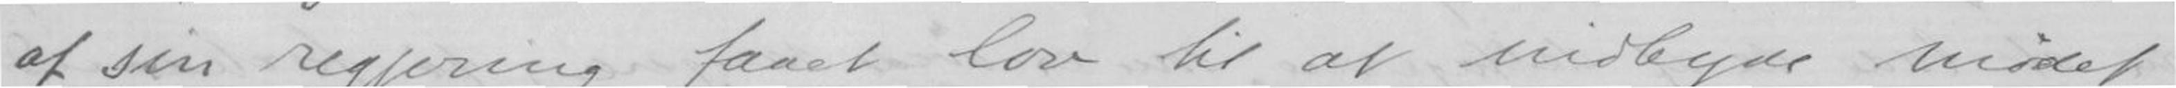af sin regjering faaet lov til at indbyde mødet
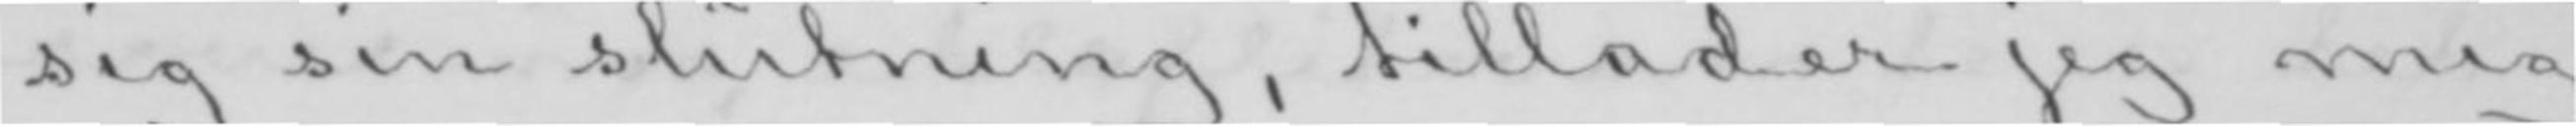sig sin slutning, tillader jeg mig
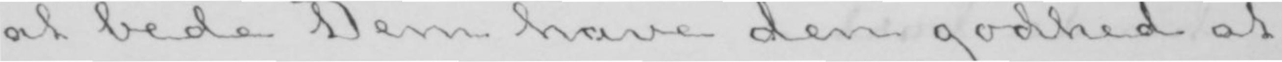at bede Dem have den godhed at
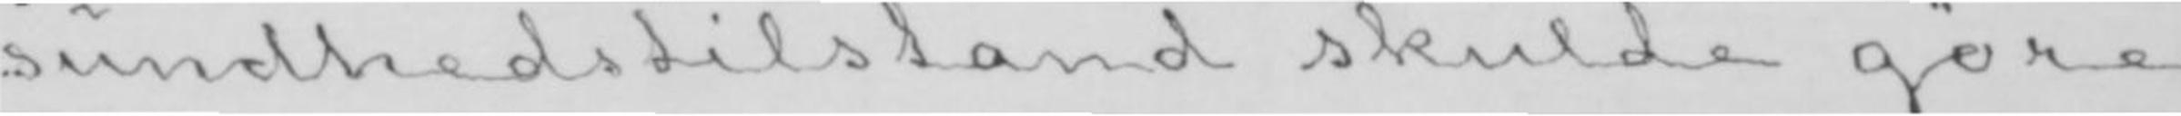sundhedstilstand skulde gøre
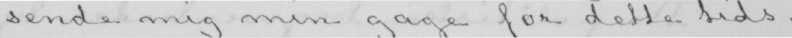sende mig min gage for dette tids-
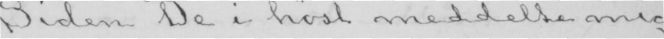Siden De i høst meddelte mig
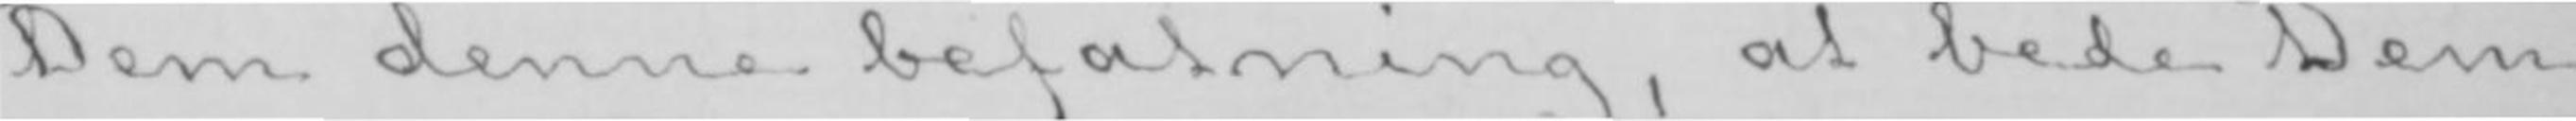Dem denne befatning, at bede Dem
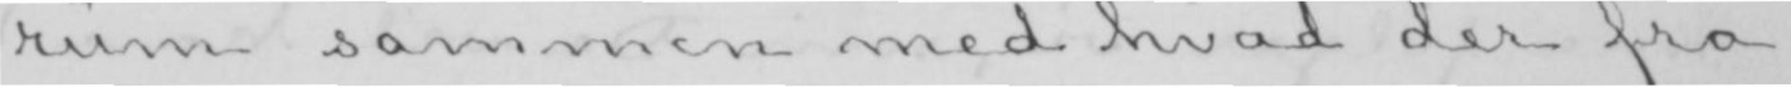rum sammen med hvad der fra
